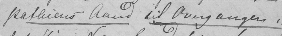pathiens Aand til Overgangen i
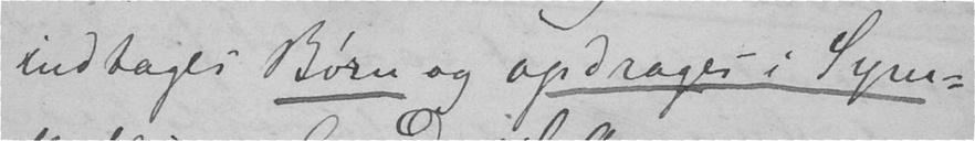indtages Børn og opdrages i Sym-
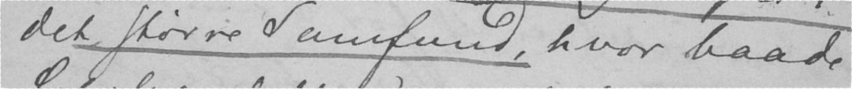det større Samfund, hvor baade
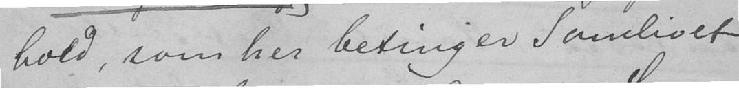hold, som her betinger Samlivet
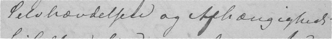Selvhævdelsen og Afhængigheds-
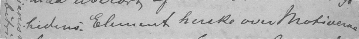hedens Element herske over Motiverne.
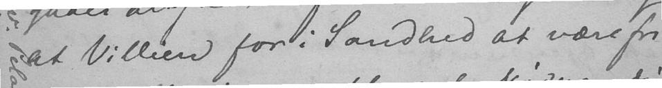at Villien for i Sandhed at være fri
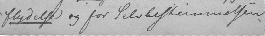flydelse og for Selvbestemmelsen
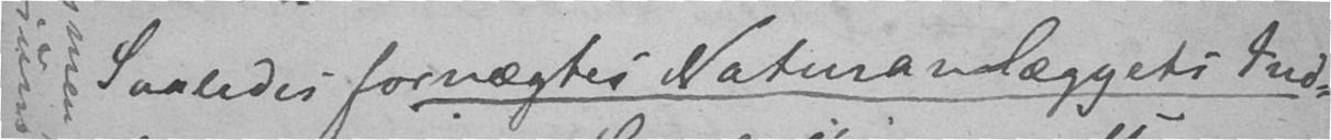Saaledes fornægtes Naturanlæggets Ind-
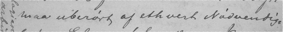maa uberørt af ethvert Nødvendig-
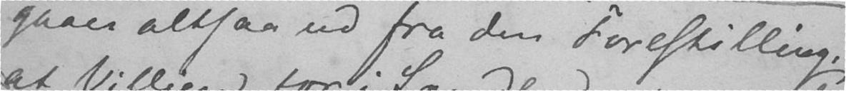gaaer altsaa ud fra den Forestilling,
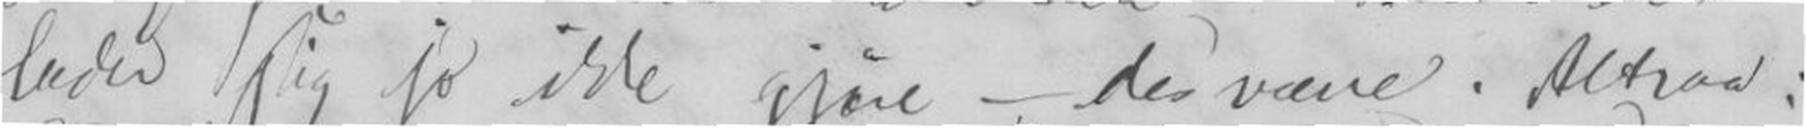lader sig jo ikke gjøre - desværre. Altsaa:
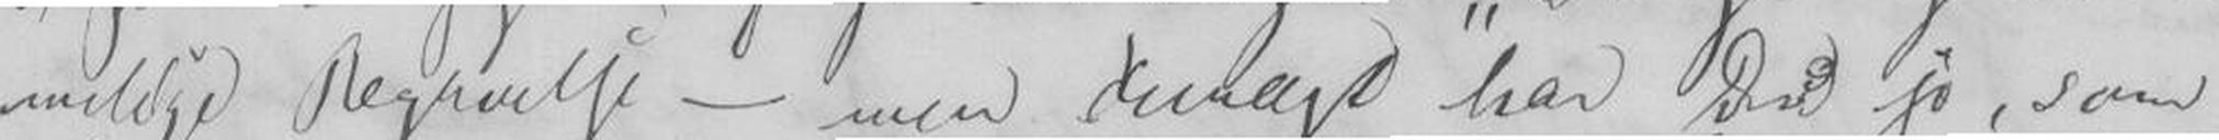melige Begavelse - men dersagt har Du jo, som
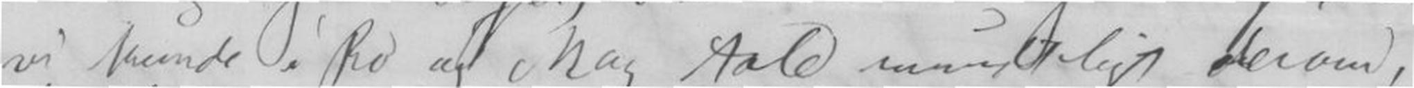vi kunde i Ro og Mag tale mundtligt derom,
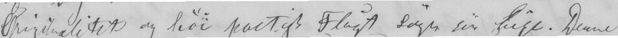Originalitet og høi poetisk Flugt Søger sin Lige. Denne
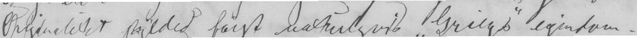Originalitet skyldes først naturligvis "Griegs" egendom-
